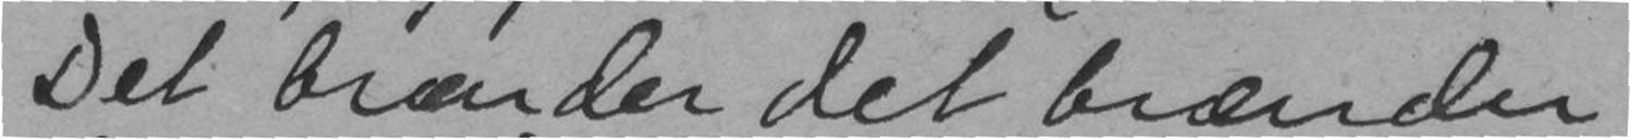Det brænder det brænder
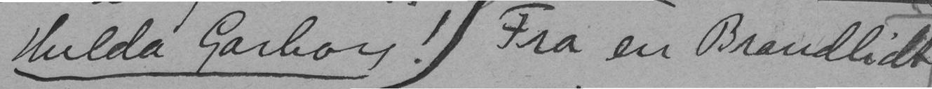Hulda Garborg! Fra en Brandlidt
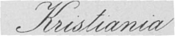Kristiania
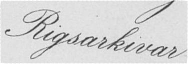Rigsarkivar
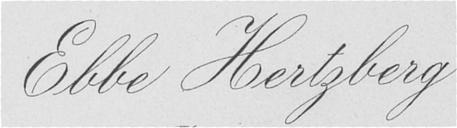Ebbe Hertzberg
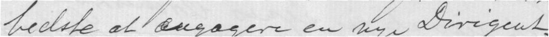bedste at ængagere en nye Dirigent
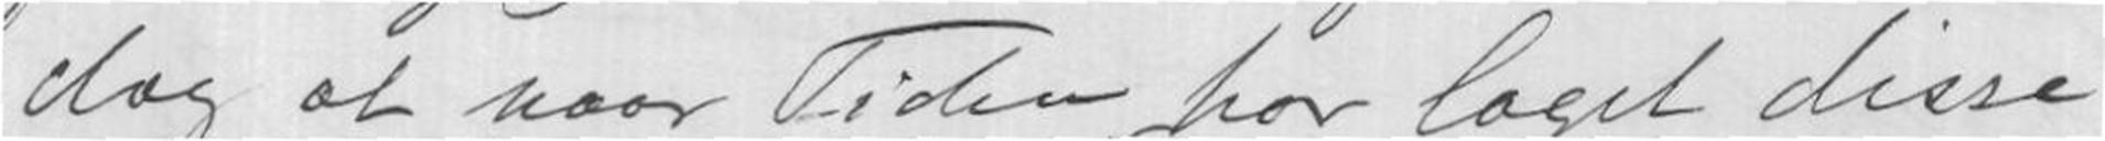dog at naar Tiden har læget disse
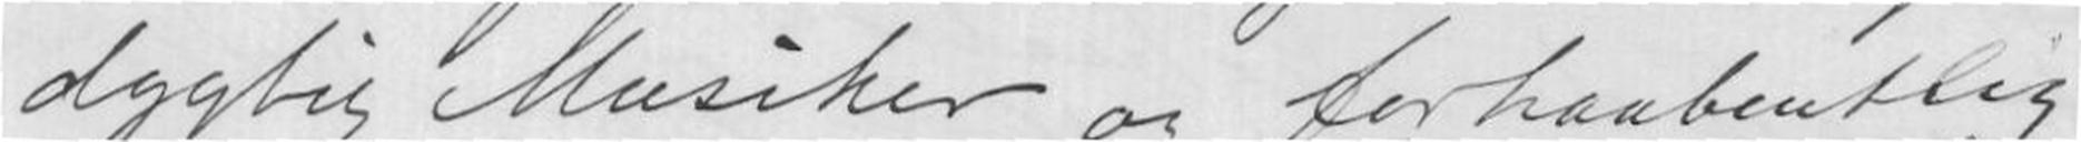dygtig Musiker og forhaabentlig
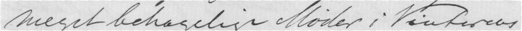meget behagelige Møder i Vinterens
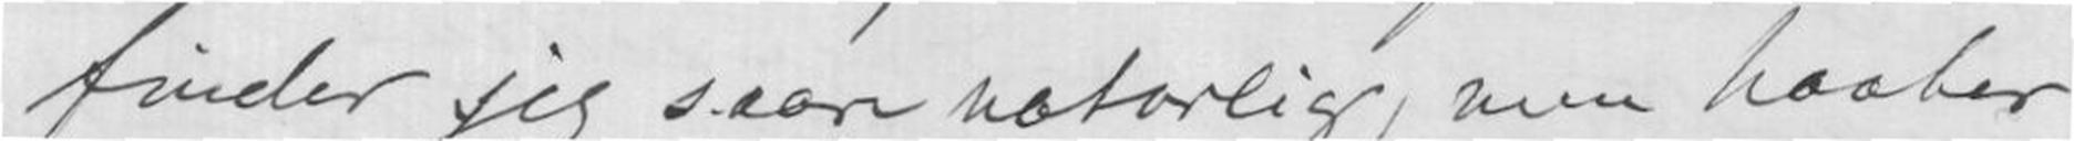finder jeg saare naturlig, men haaber
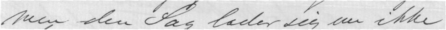men den Sag lader sig nu ikke
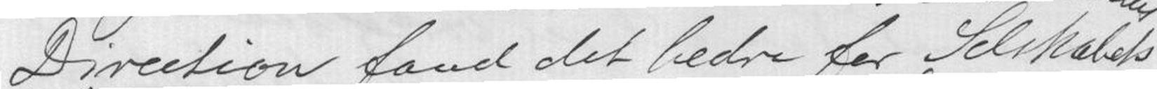Direction fand det bedre for Selskabets
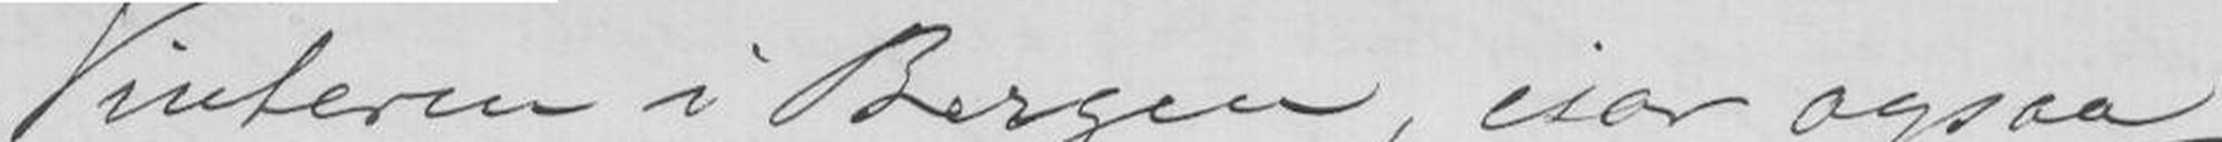Vinteren i Bergen, især ogsaa
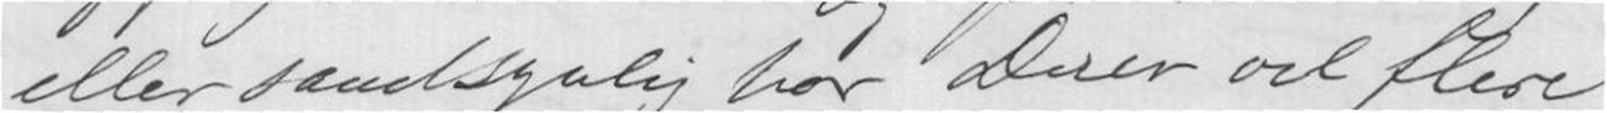eller sandsynlig har Derer vel flere
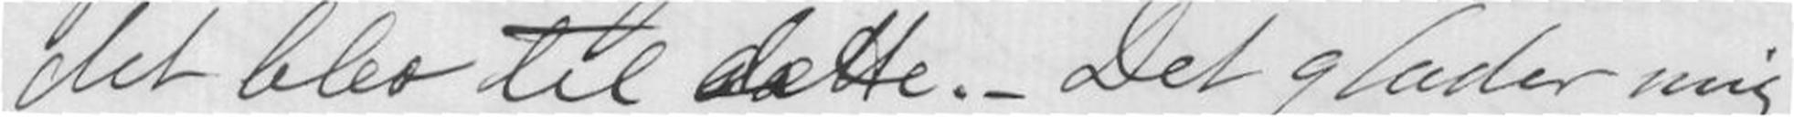det blev til dette. - Det glæder mig
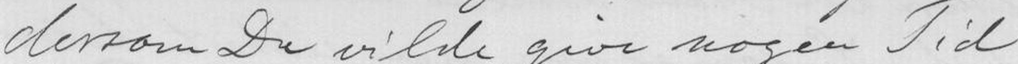dersom Du vilde give nogen Tid
